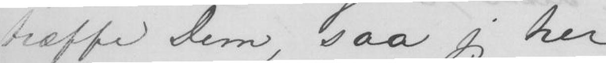træffe Dem, saa jeg her
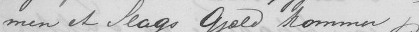men et Slags Gjæld kommer jeg
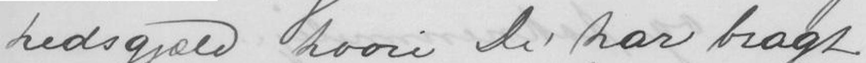hedsgjæld hvori De har bragt
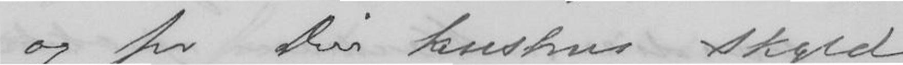og for Din hustrus skyld.
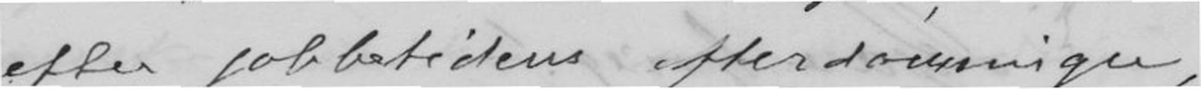efter jobbetidens efterdønninger,
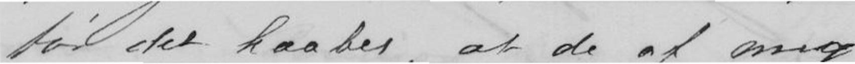tør det haabes, at de af mig
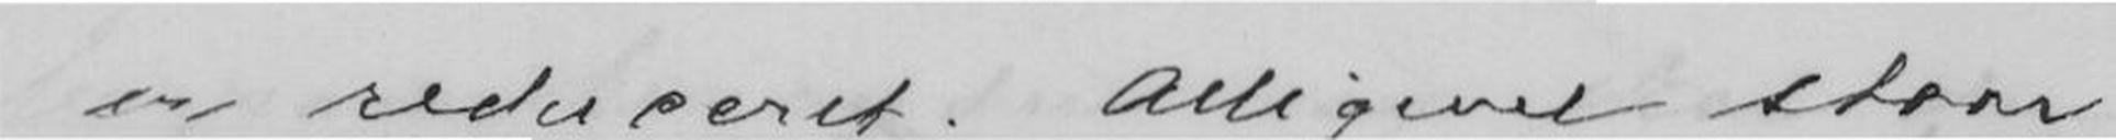er reduceret. Alligevel staar
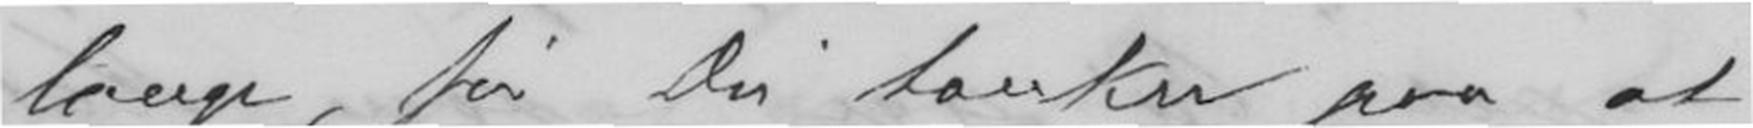længe, før Du tænker paa at
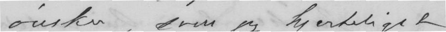ønsker, som jeg hjerteligst
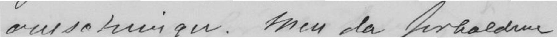omsetninger. Men da forholdene
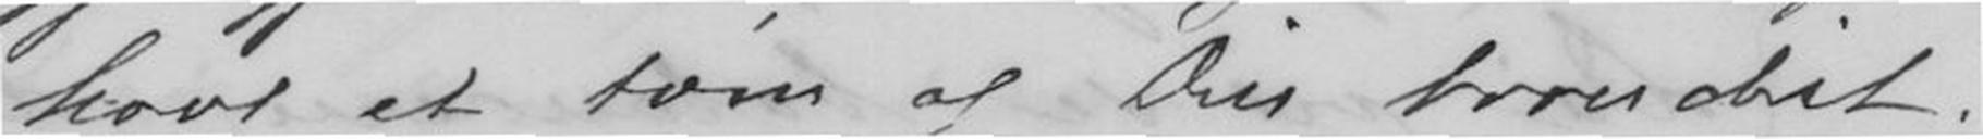havt et tørn af Din bronchit.
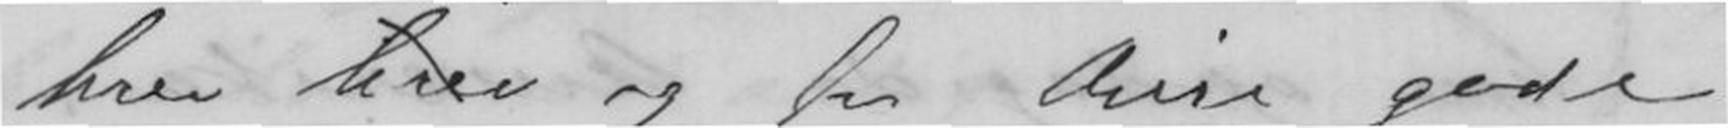brev og for Dine gode
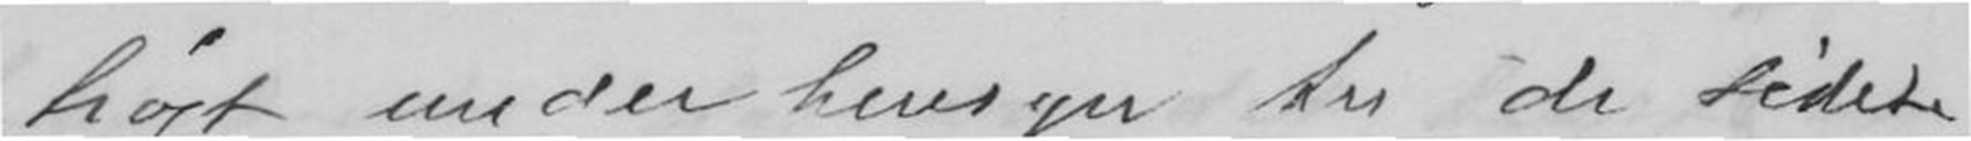høgt under hensyn til de sidste
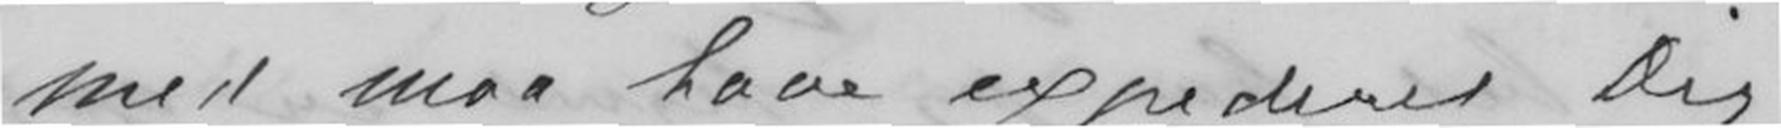med maa have expederes Dig
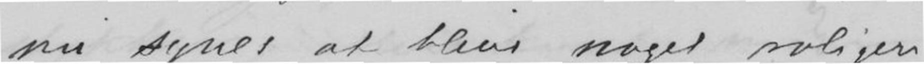nu synes at blive noget roligere
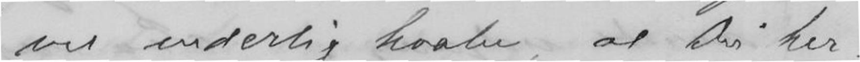vil endelig haabe at Du her-
